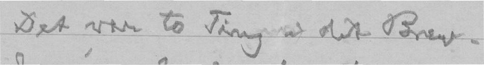Det var to Ting i dit Brev.
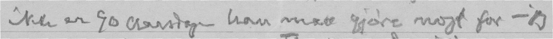ikke er 90 Aarsdagen han maa gjøre nogt for - jeg
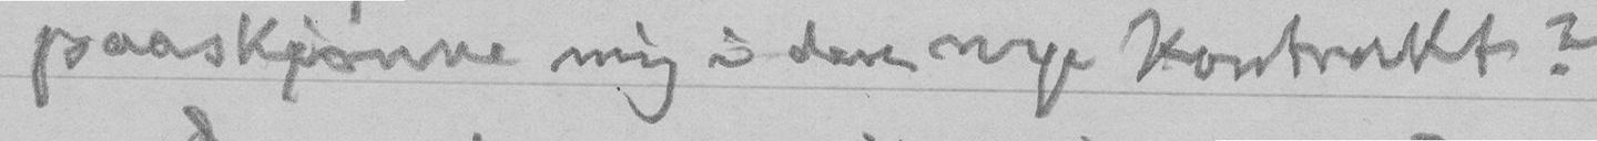paaskjønne mig i den nye Kontrakt?
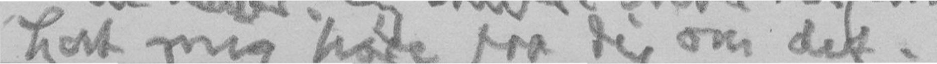Lat mig høre fra dig om det.
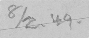8/2. 49.
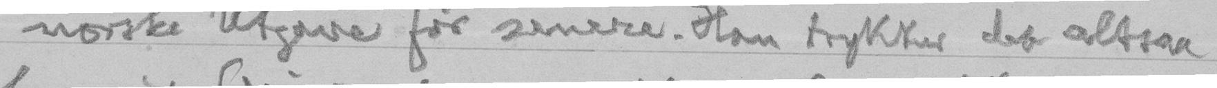norske Utgave før senere. Han trykker det altsaa
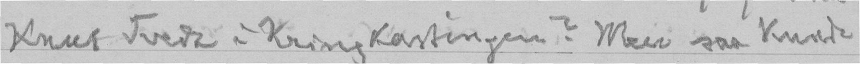Knut Tvedt i Kringkastingen? Men saa kunde
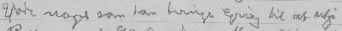gjøre noget som kan tvinge Grieg til at utgi
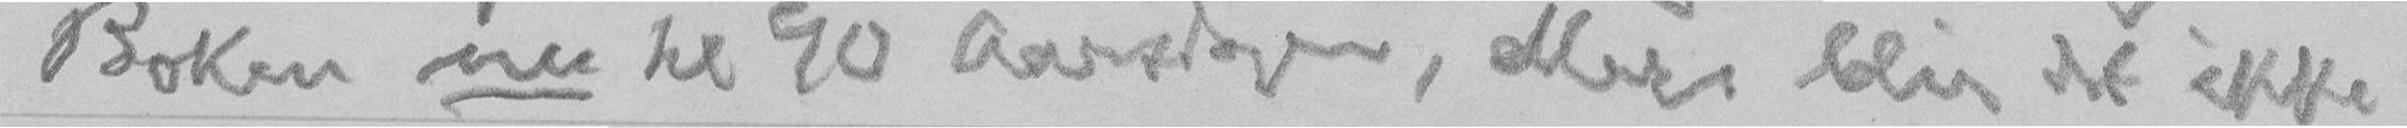Boken nu til 90 Aarsdagen, ellers blir det ikke
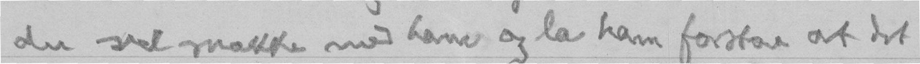du vel snakke med ham og la ham forstaa at det
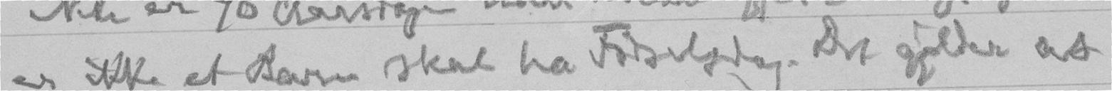er ikke et Barn skal ha Fødselsdag. Det gjelder at
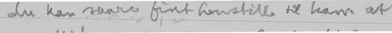du kan saare fint henstille til ham at
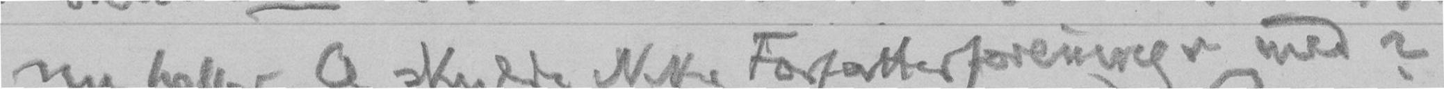nu heller. Og skulde ikke Fortatterforeningen med?
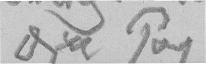Din Pap
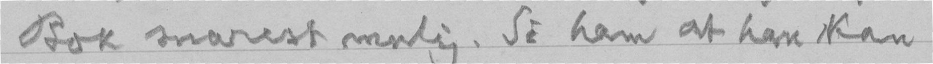Bok snarest mulig. Si ham at han kan
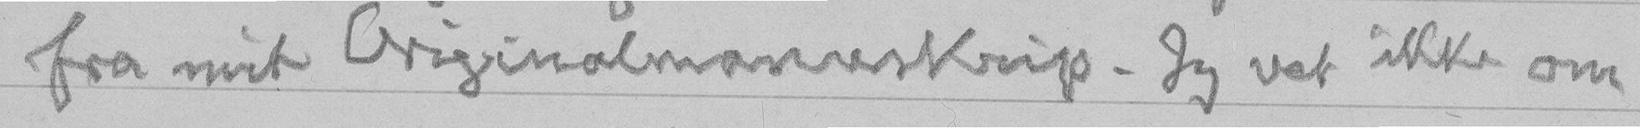fra mit Originalmanuskrip. Jeg vet ikke om
